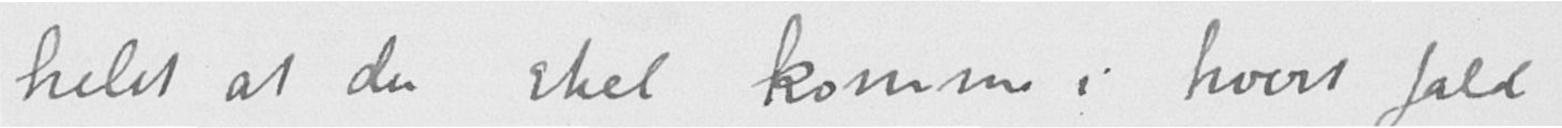helst at du skal komme i hvert fald
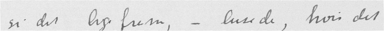si det lige frem, - lusede, hvis det
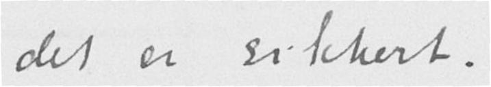det er sikkert.
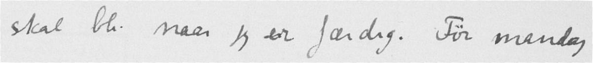skal bli naar jeg er færdig. Før mandag
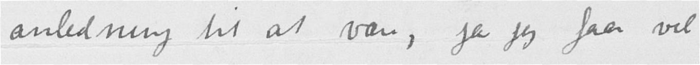anledning til at være, ja jeg faar vel
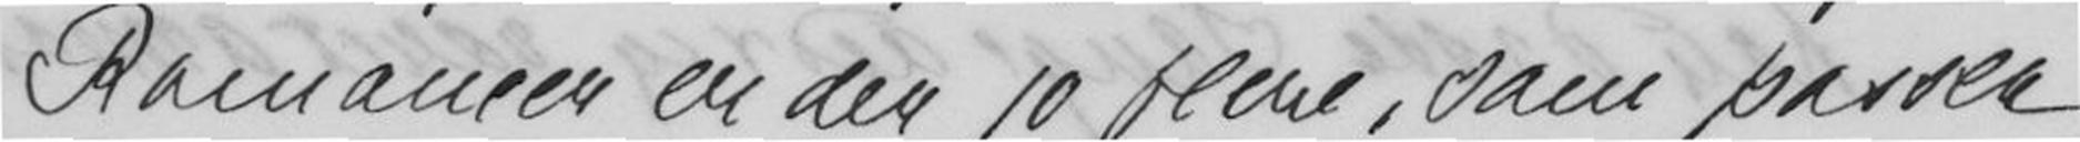Romancer er der jo flere, som passer
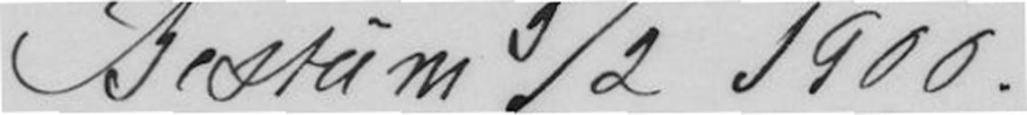Bestum 5/2 1900
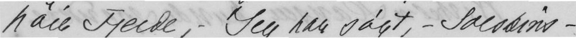høie Fjelde,- jeg har søgt,- Solskins-
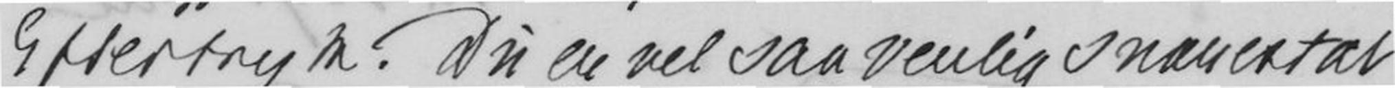Eftertryk. Du er vel saa venlig snarest at
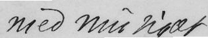med muliget
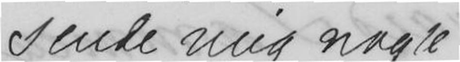sende mig nogle
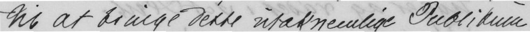til at bruge Dette utaknemlige Publikum
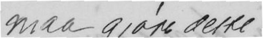maa gjøre dette
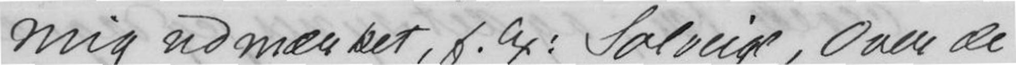mig udmærket, f.ex. Solveig, over de
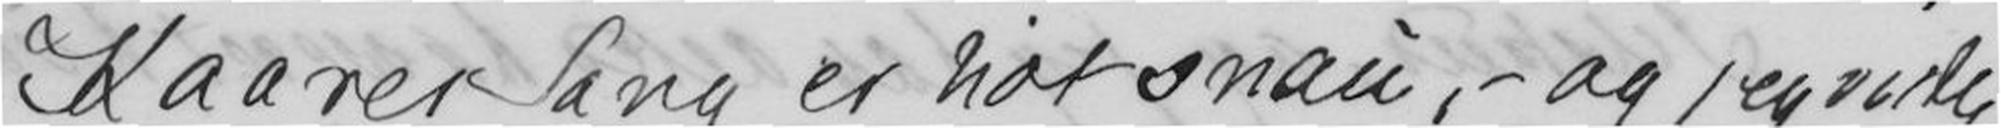Kaares Sang er hørsnau,- og jeg vilde
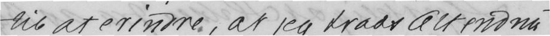til at erindre, at jeg trods Alt endnu
